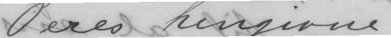Deres hengivne
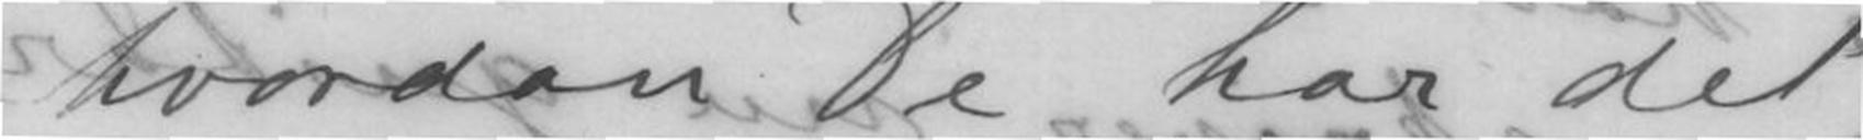hvordan De har det
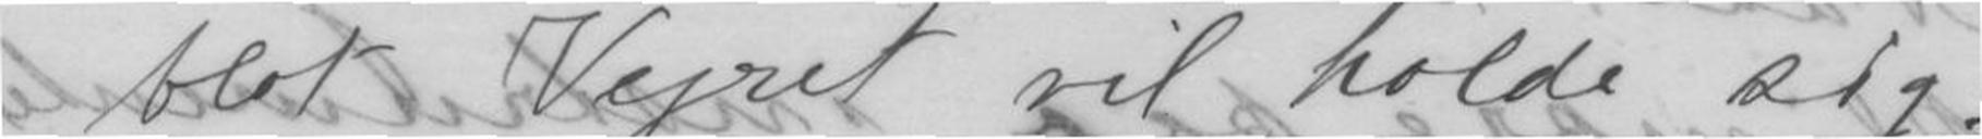blot Vejret vil holde sig.
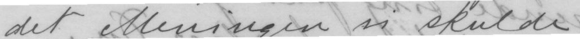det Meningen vi skulde
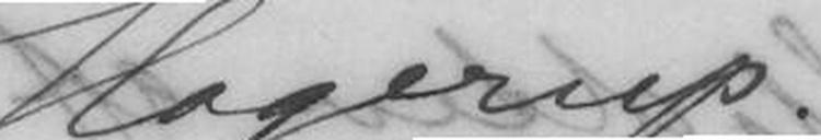Hagerup
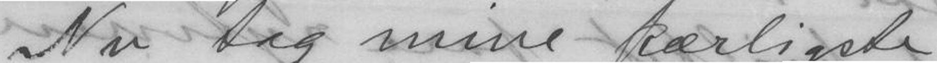Nu tag mine kærligste
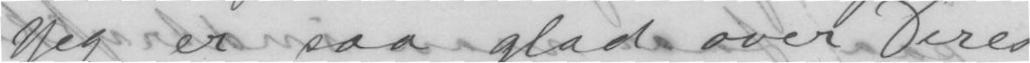Jeg er saa glad over Deres
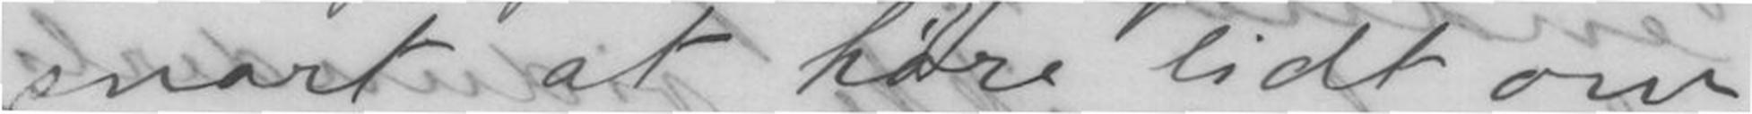snart at høre lidt om
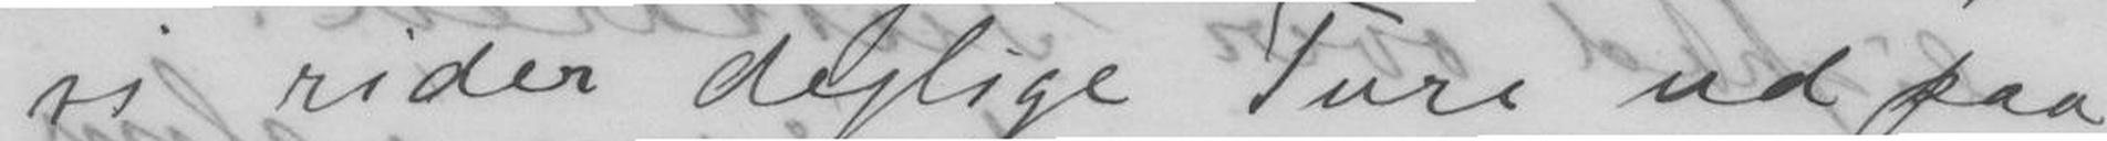vi rider dejlige Ture ud paa
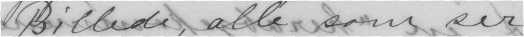Billede, alle som ser
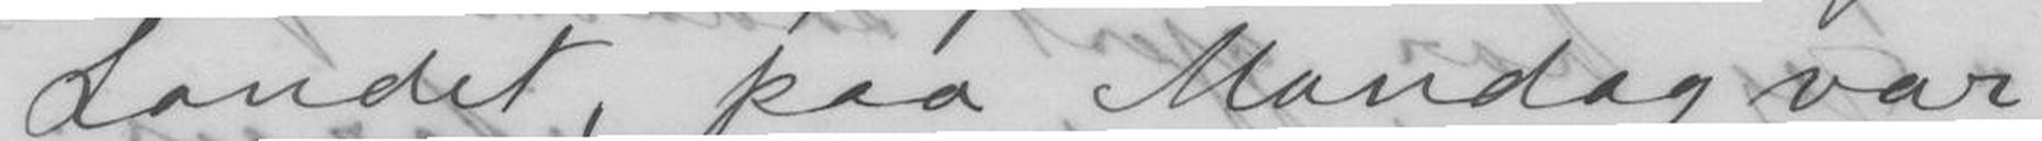Landet, paa Mandag var
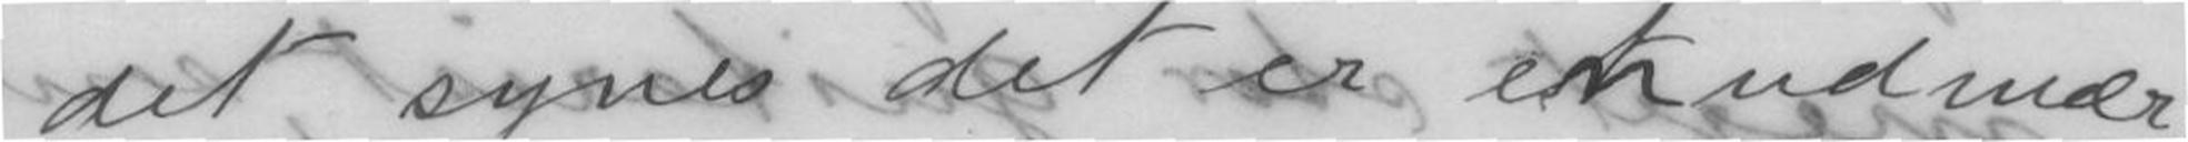det synes det er en udmær-
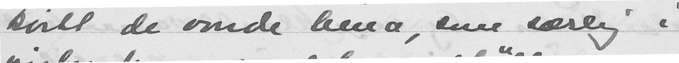kvitt de vonde bena, som særlig i
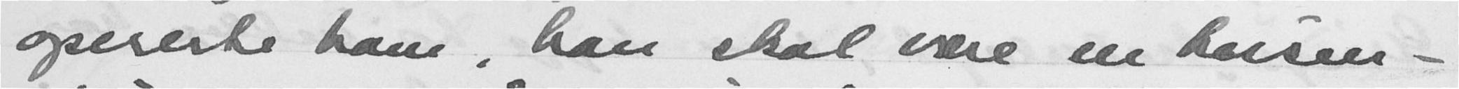opererte ham, han skal være en tusen-
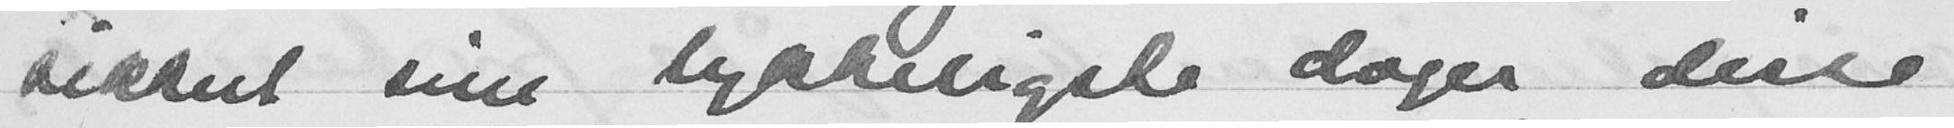sikkert sine lykkeligste dager disse
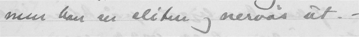men han ser sliten og nervøs ut. -
